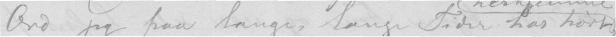Ord jeg paa lange, lange Tider har hørt,
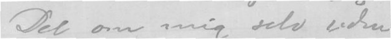Del om mig selv uden
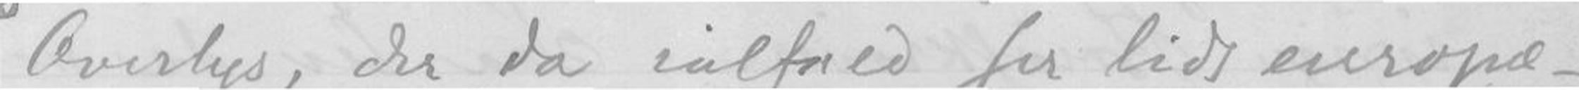Overlys, der da ialfald ser lidt europæ-
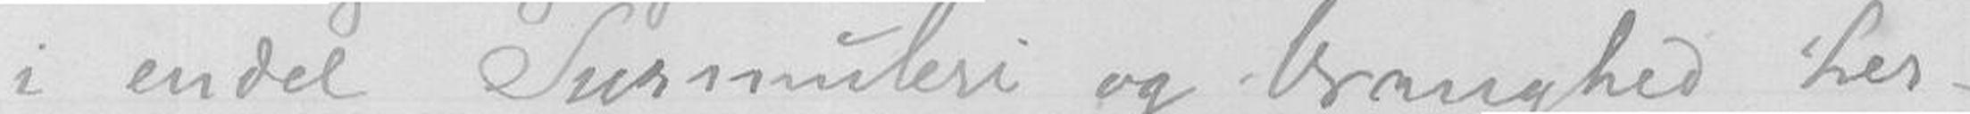i endel Surmuleri og vranghed her-
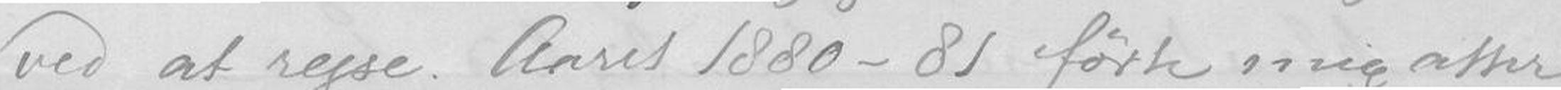ved at rejse. Aaret 1880-81 førte mig atter
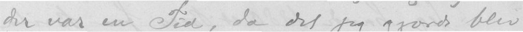der var en Tid, da det jeg gjorde blev
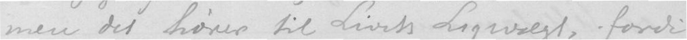men det hører til Livets Ligevægt, fordi
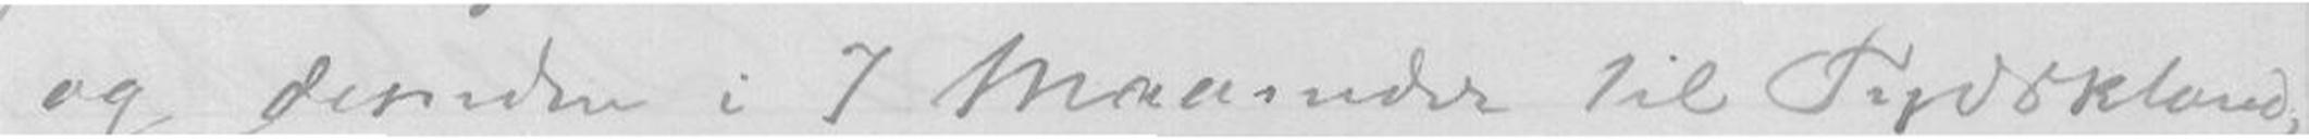og desuden i 7 Maaneder til Tydskland,
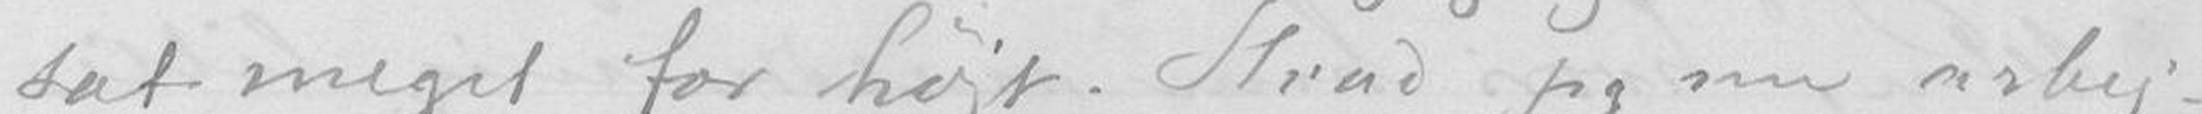sat meget for højt. Hvad jeg nu arbej-
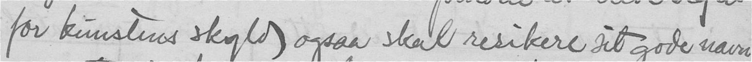for kunstens skyld ogsaa skal resikere det gode havn
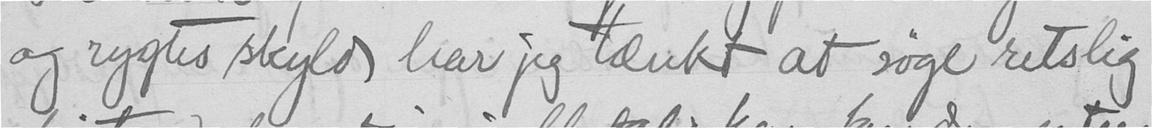og rygtes skulds har jeg tænkt at søge retslig
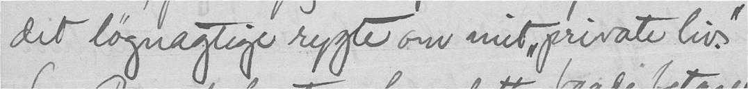det løgnagtige rygte om mit "private liv."
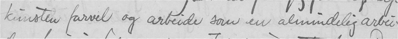kunsten farvel og arbeide som en almindelig arbei-
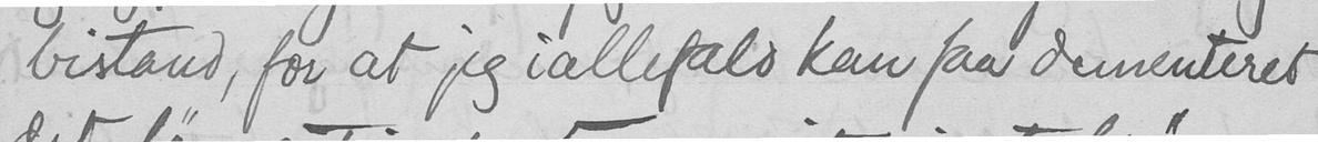bistand, for at jeg iallefald kan faa dementeret
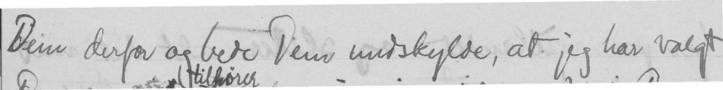Dem derfor og bede Dem undskylde, at jeg har valgt
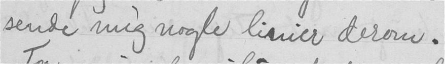sende mig nogle linier derom.
